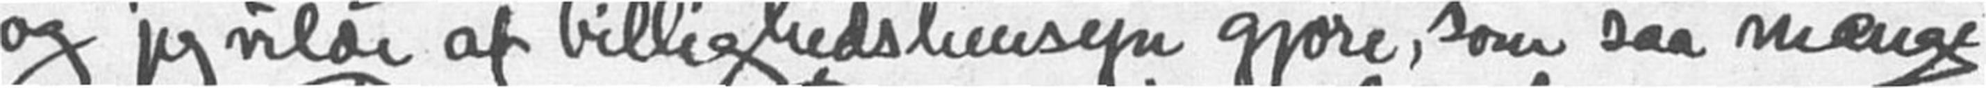og jeg vilde af billighedshensyn gjøre, som saa mange
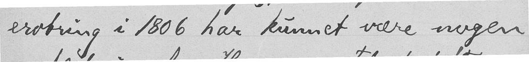erobring i 1806 har kunnet være nogen
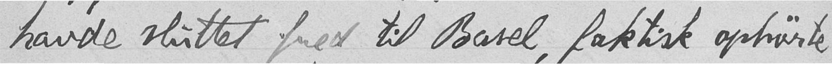havde sluttet fred til Basel, faktisk ophørte
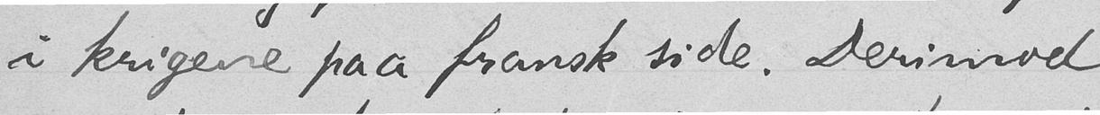i krigene paa fransk side. Derimod
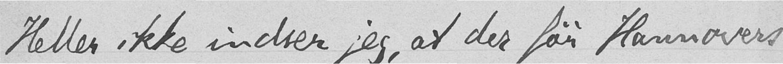Heller ikke indser jeg, at der før Hannovers
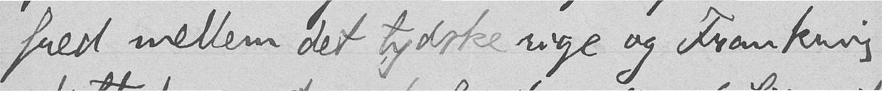fred mellem det tydske rige og Frankrig
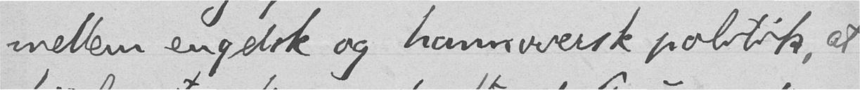mellem engelsk og hannoversk politik, at
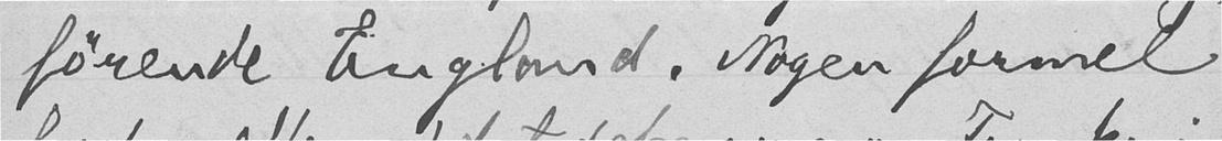førende England. Nogen formel
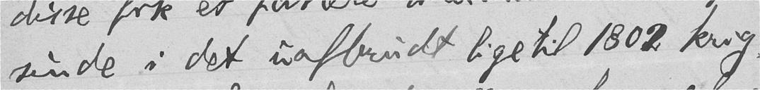sinde i det uafbrudt ligetil 1802 krig-
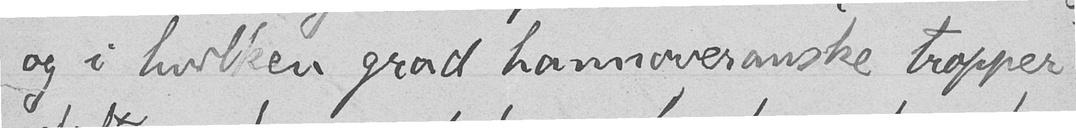og i hvilken grad hannoveranske tropper
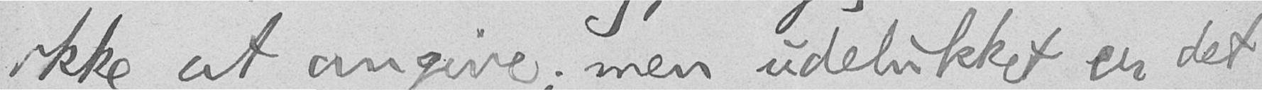ikke at angive; men udelukket er det
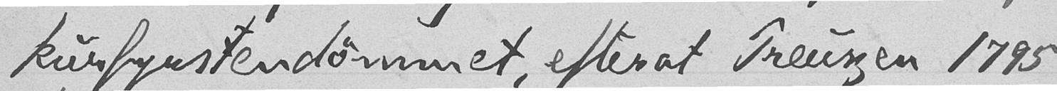kurfyrstendømmet, efterat Preuszen 1795
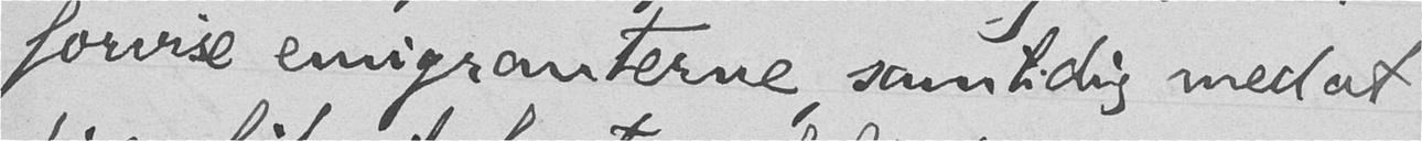forvise emigranterne, samtidig med at
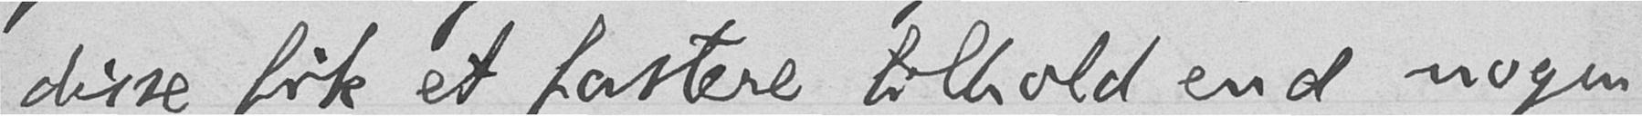disse fik et fastere tilhold end nogen-
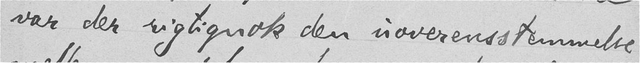var der rigtignok den uoverensstemmelse
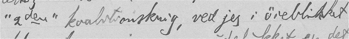"2den" koalitionskrig, ved jeg i øieblikket
